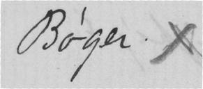Bøger. x
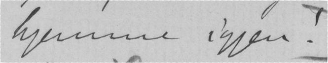hjemme igjen!
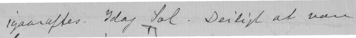igaaraftes. Idag Sol. Deiligt at være
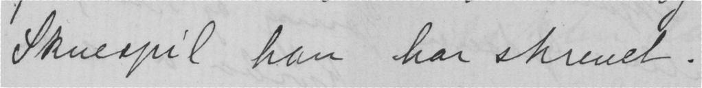Skuespil han har skrevet.
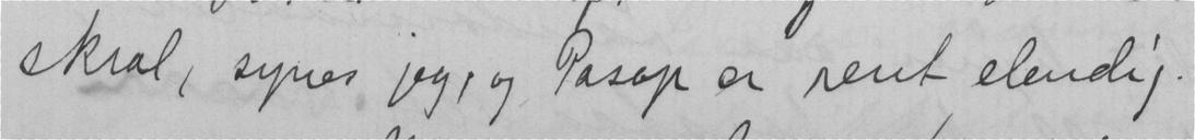skral, synes jeg, og Pasop er rent elendig.
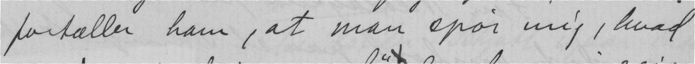fortæller ham, at man spør mig, hvad
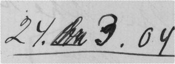24. 3.04
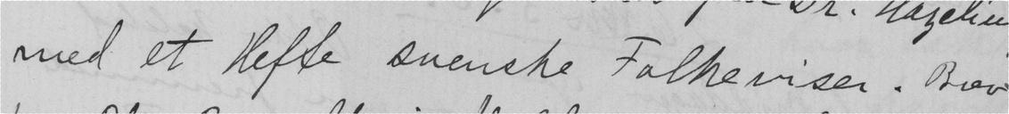med et Hefte svenske Folkeviser. Brev
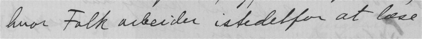hvor Folk arbeider istedetfor at læse
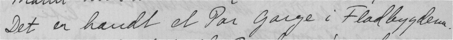Det er hændt et Par Gange i Fladbygdene.
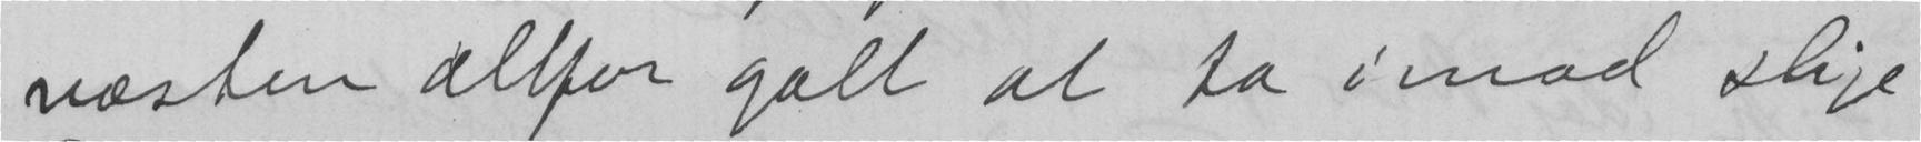næsten altfor galt at ta imod slige
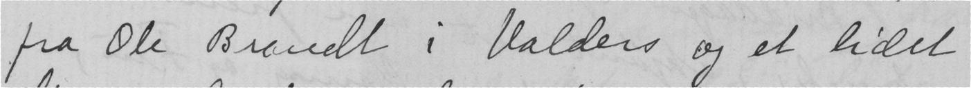fra Ole Brandt i Valders og et lidet
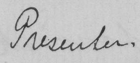Presenter.
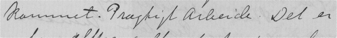kommet. Prægtigt Arbeide. Det er
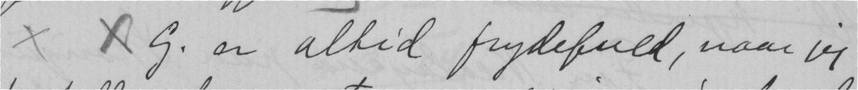x x G. er altid frydefuld, naar jeg
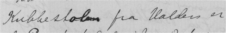Kubbestolen fra Valders er
